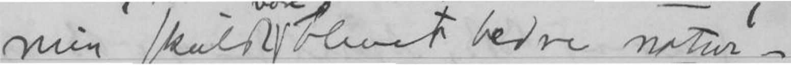min skulde blevet bedre natur-
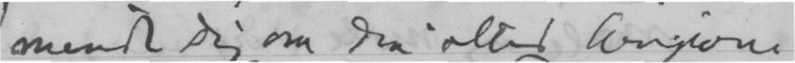mindt Dig, om Din aller hengivne
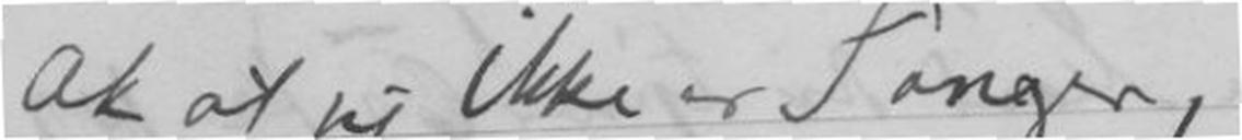Ak at jeg ikke er Sanger,
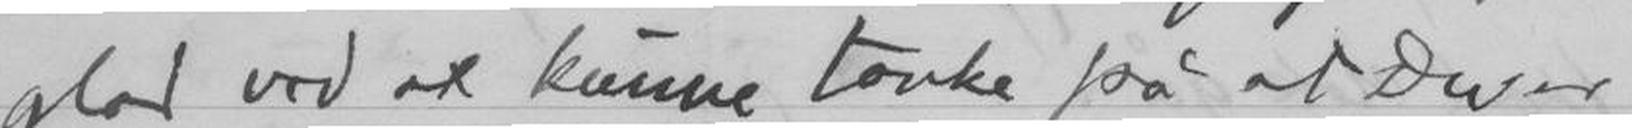glad ved at kunne tænke på at Du er
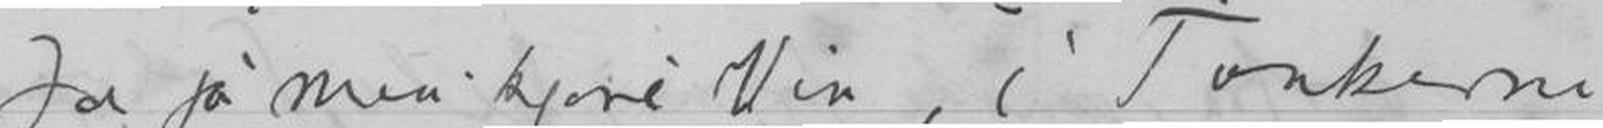Ja ja min kjære Ven, i Tankerne
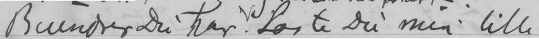Beundrer Du har Læste Du min lille
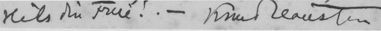Hils din Frue! - Knud Claussen.
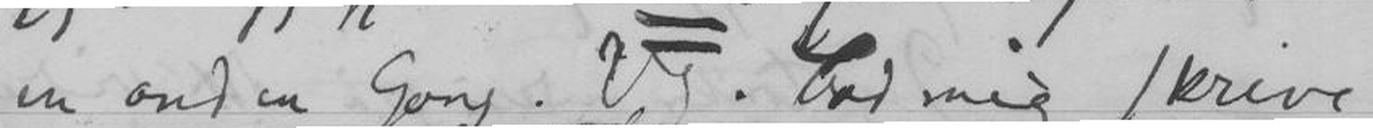en anden Gang. VG bad mig skrive
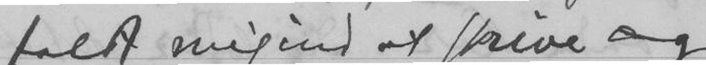faldt mig ind at skrive og
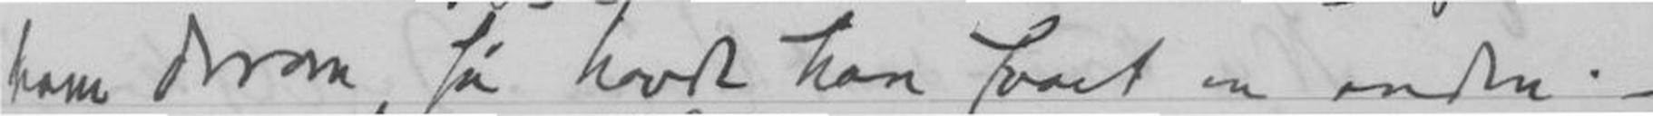ham derom, så havde han svart en anden ; -
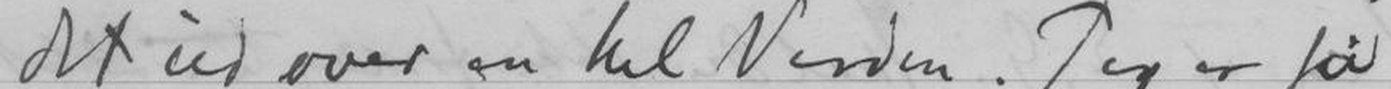det ud over en hel Verden. Jeg er så
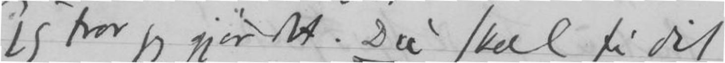Jeg tror jeg gjør det Du skal få dit
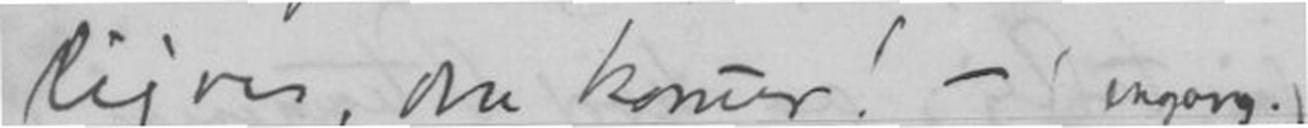ligvis, den komer! - (engang).
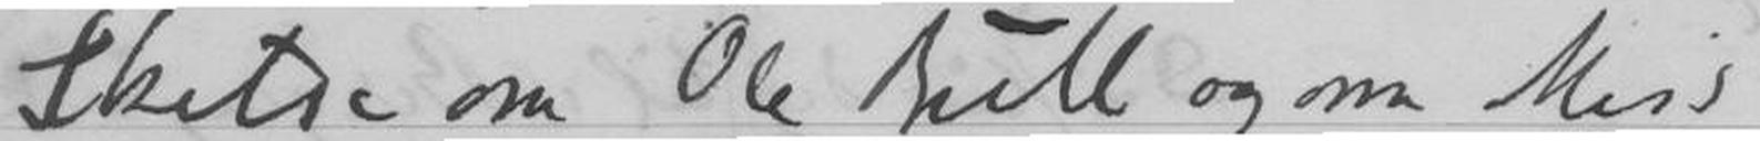Sketse om Ole Bull og om Miss
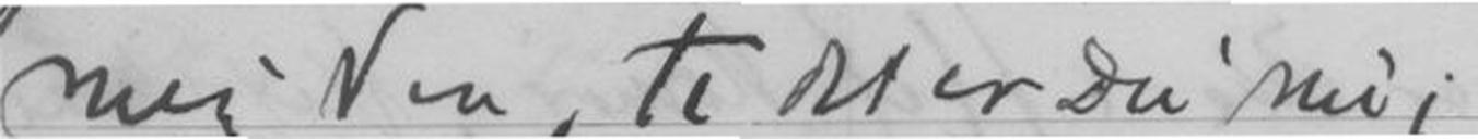min Ven, te det er Du nu;
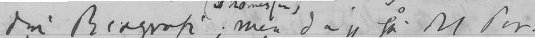din Biografi ; men da jeg så dit Por-
